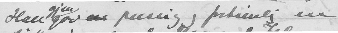Han gjen/gav  pussig og fortsenlig en
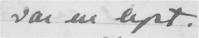var en lyst.
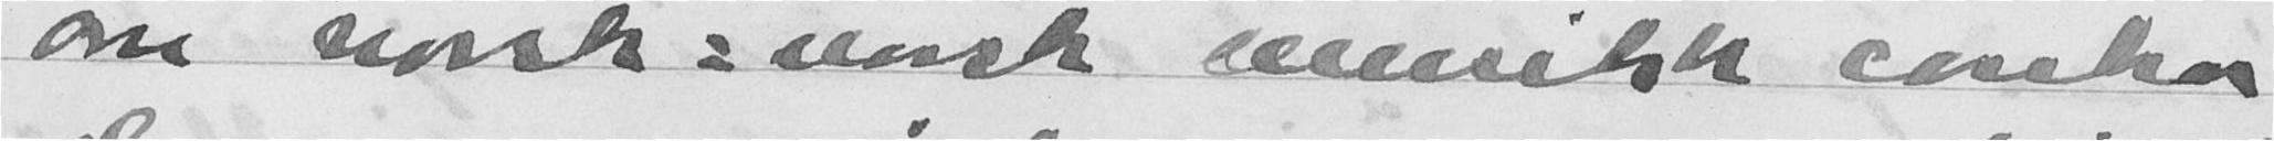om norsk-isevisk musikk cossko
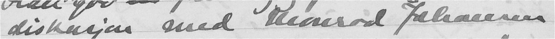diskusjon med Monrad Johansen
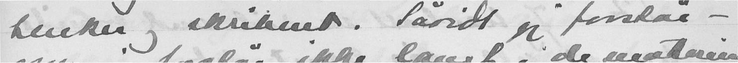tenker og skribent. Såvidt jeg forstår -
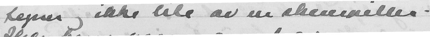tegner og ikke lite av en skuespiller.
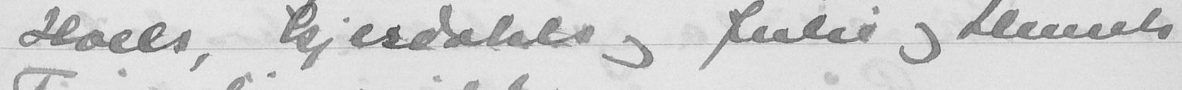Hoels, Gjesdahls og Julie og Henrik
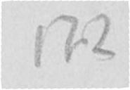172
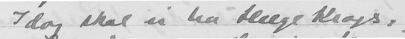I dag skal vi ha Helge Krogs,
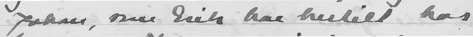Johan, som Erik har bestilt hos
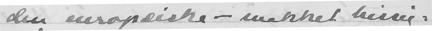den europeiske - snakket hissig
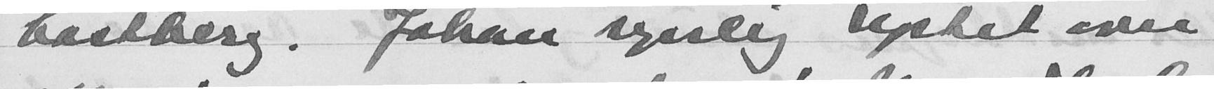Castberg. Johan synlig rystet over
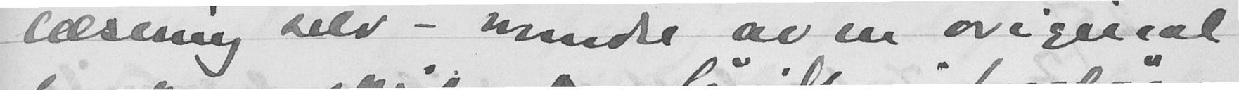læsning selv - mindre av en original
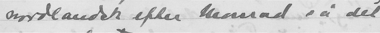nordlandsk efter Monrad sa det
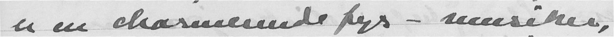er en charmerende fyr - susikker,
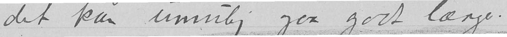det kan umulig gaa godt længe.
